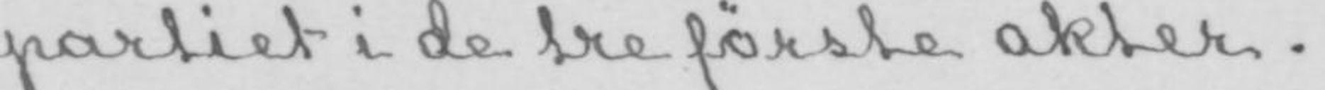partiet i de tre første akter.
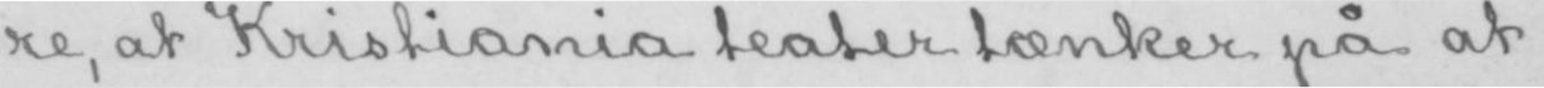re, at Kristiania teater tænker på at
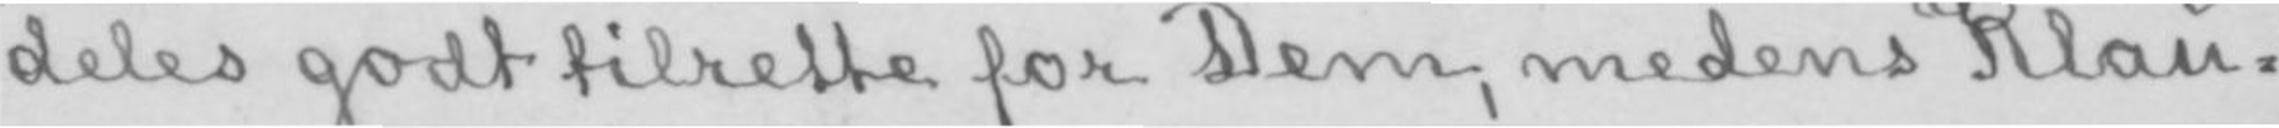deles godt tilrette for Dem, medens Klau-
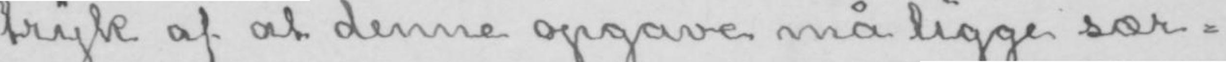tryk af at denne opgave må ligge sær-
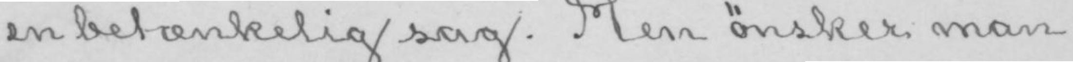en betænkelig sag. Men ønsker man
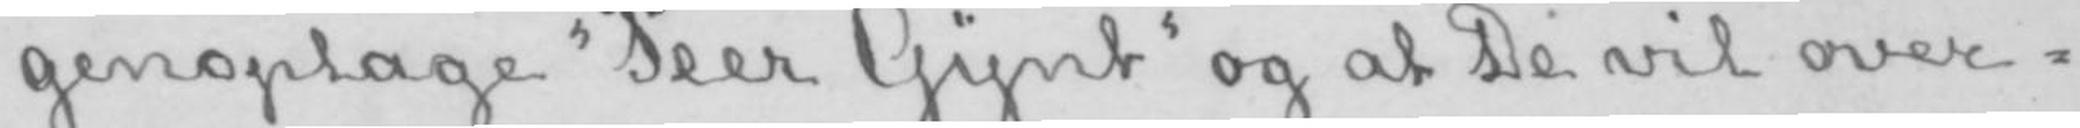genoptage «Peer Gynt» og at De vil over-
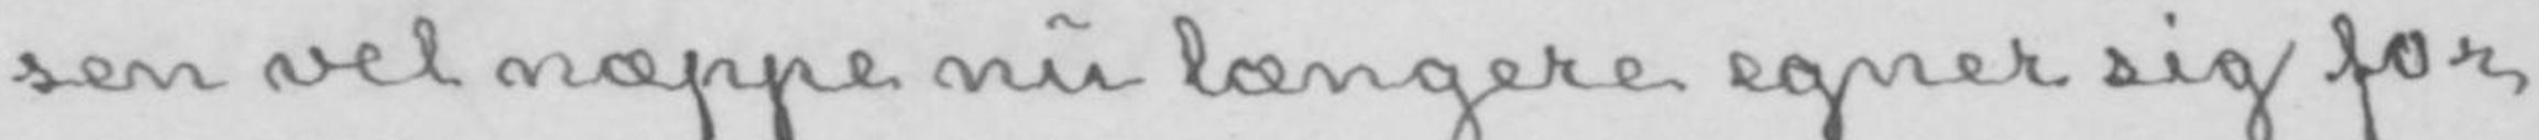sen vel næppe nu længere egner sig for
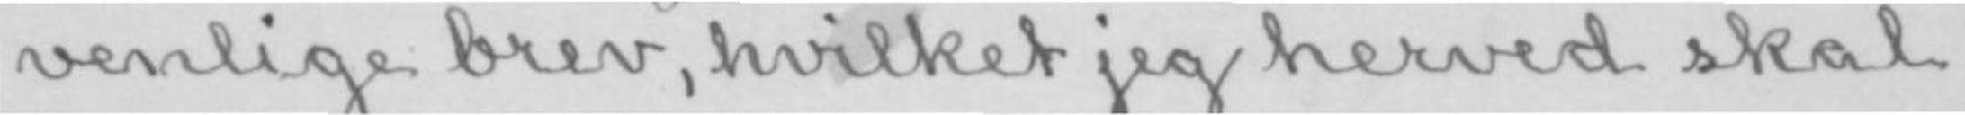venlige brev, hvilket jeg herved skal
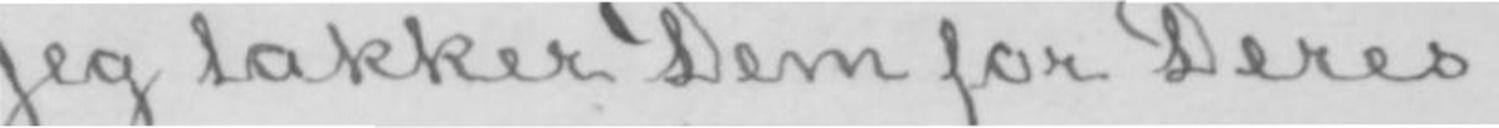Jeg takker Dem for Deres
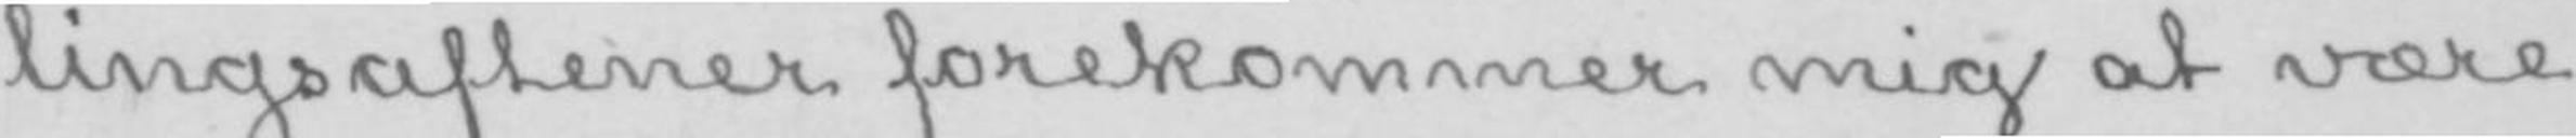lingsaftener forekommer mig at være
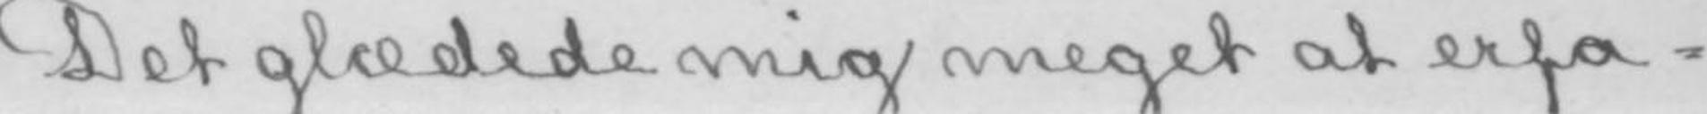Det glædede mig meget at erfa-
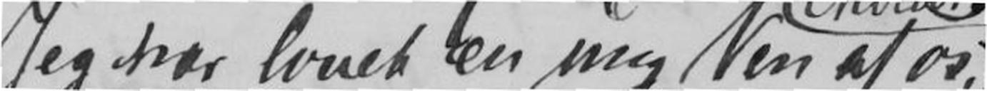Jeg har lovet en ung Ven af os,
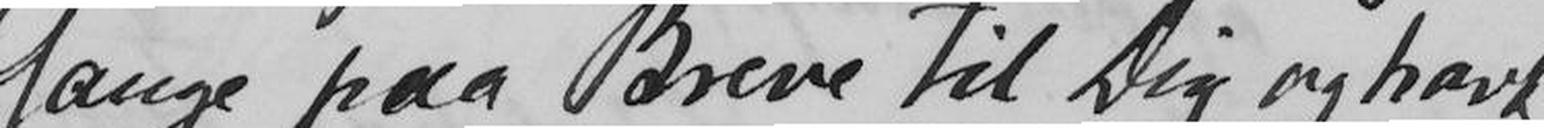Gange paa Breve til Dig og havt
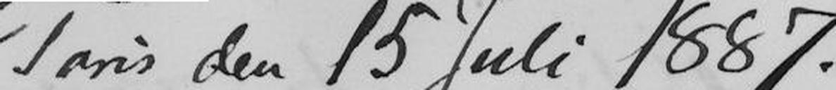Paris den 15 Juli 1887.
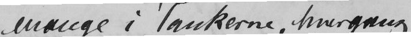mange i Tankerne, hvergang
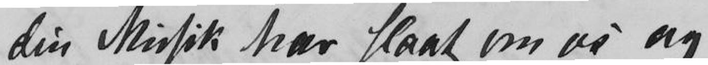din Musik har slaat om os og
